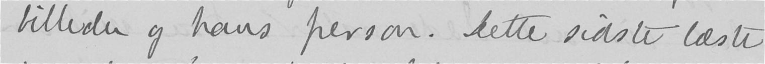billeder og hans person. Dette sidste læste
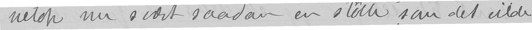netop nu svært saadan en støtte som det vilde
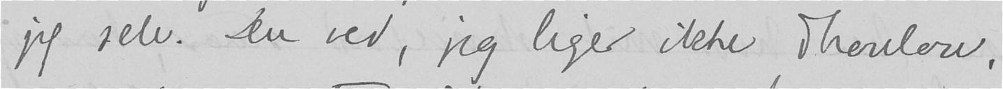jeg selv. Du ved, jeg liger ikke Thaulow,
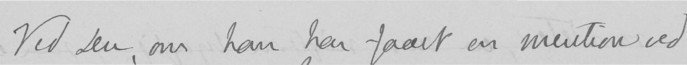Ved Du, om han har faaet en mention ved
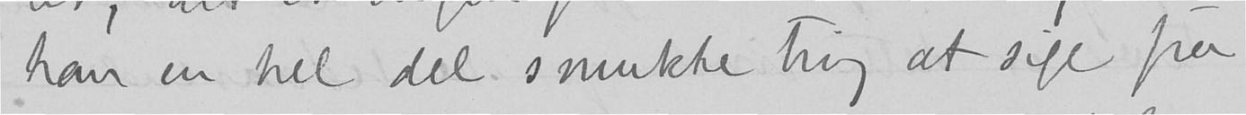han en hel del smukke ting at sige fra
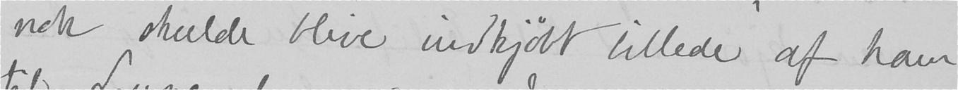nok skulde blive indkjøbt billede af ham
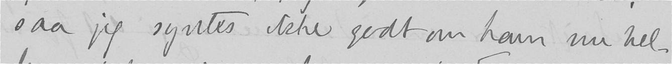saa jeg syntes ikke godt om ham nu hel-
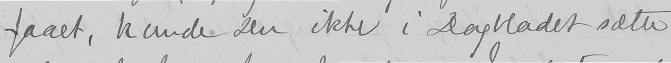faaet, kunde Du ikke i Dagbladet sætte
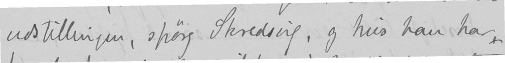udstillingen, spørg Skredsvig, og hvis han har
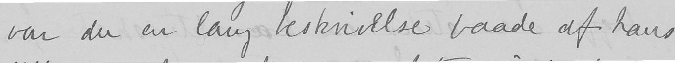var der en lang beskrivelse baade af hans
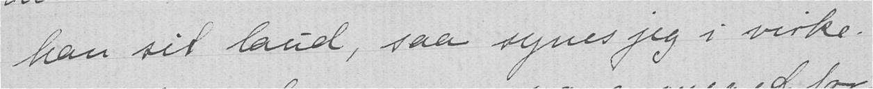han sit laud, saa synes jeg i virke-
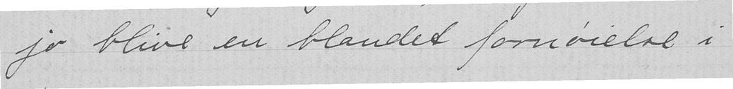jo blive en blandet fornøielse i
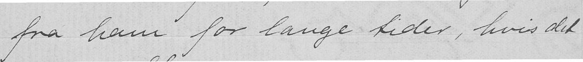fra ham for lange tider, hvis det
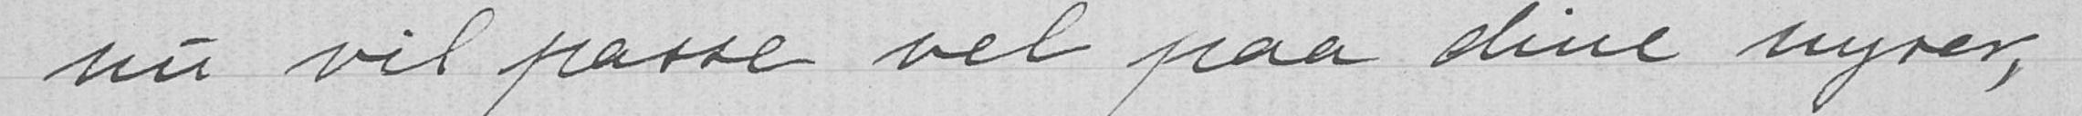nu vil passe vel paa dine nyrer,
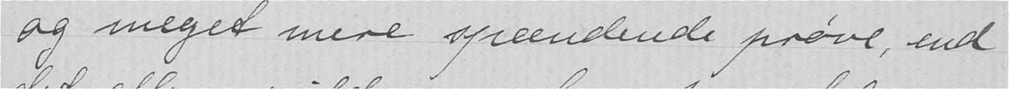og meget mere spændende prøve, end
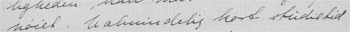nøiet. Ualmindelig kort studietid
